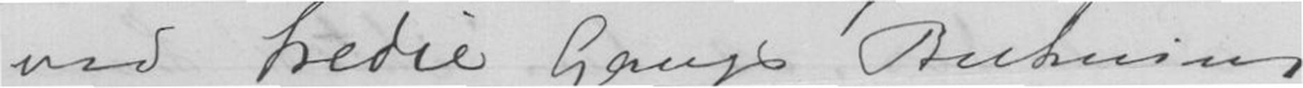ved tredie Gangs Bukning
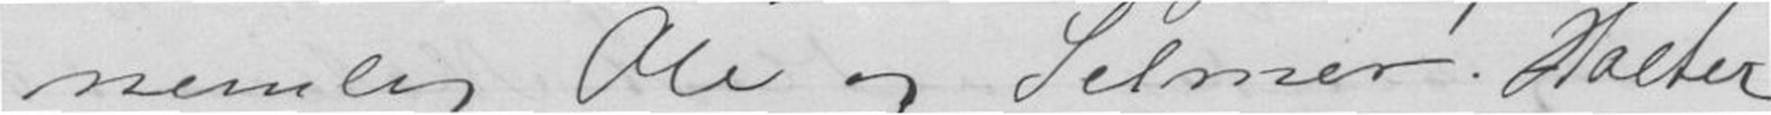nemlig Ole og Selmer. Holter
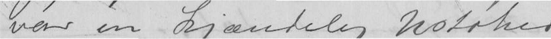var en kjændelig Notøhed
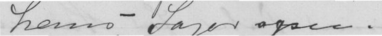hams Sager ogsaa.
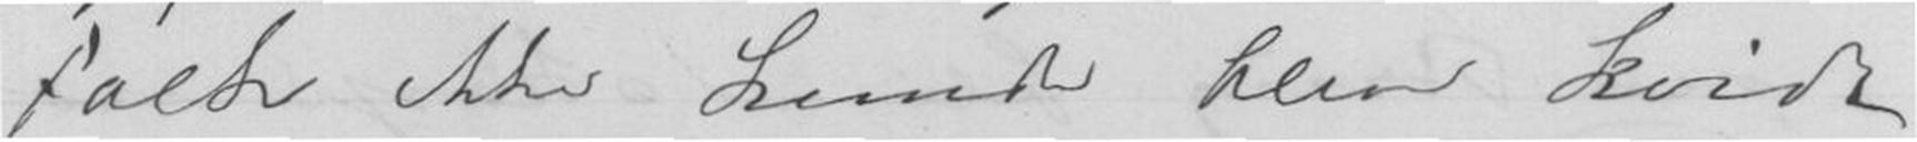Folk ikke kunde blive kvidt
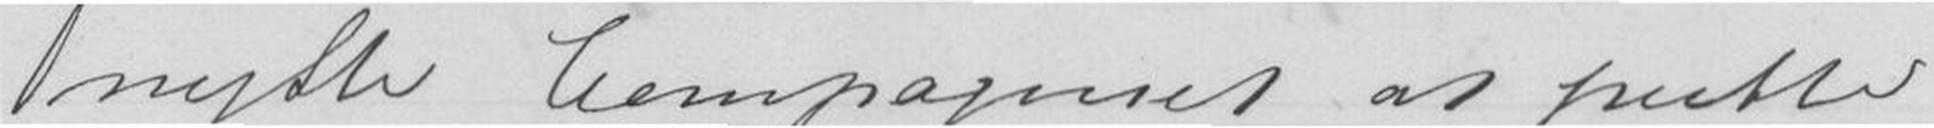nytte Campagenet at putte
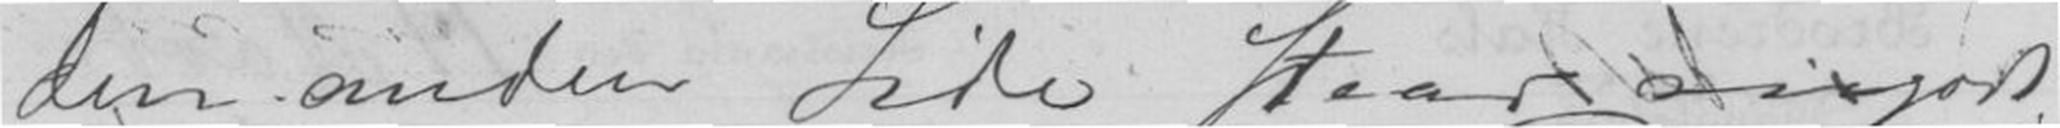den anden Side staar saagodt
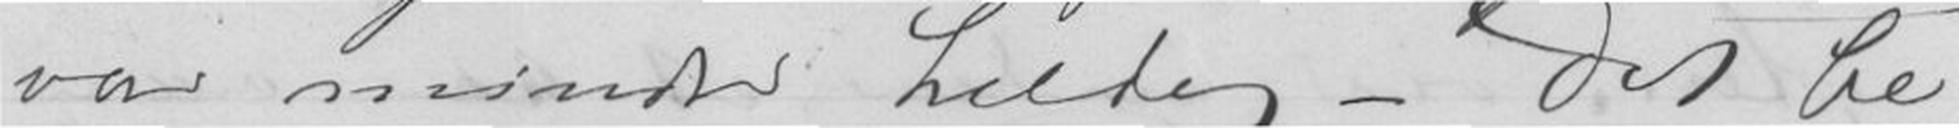var mindre heldig - Det be
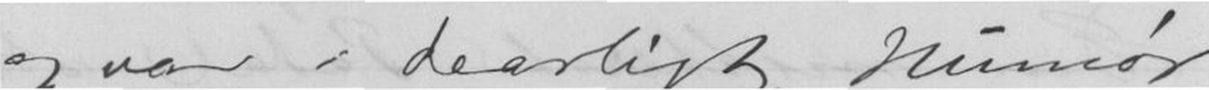og var i daarligt Humør
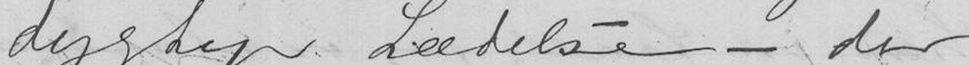dygtige Lædelse - der
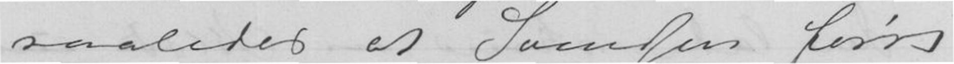saaledes at Svendsen først
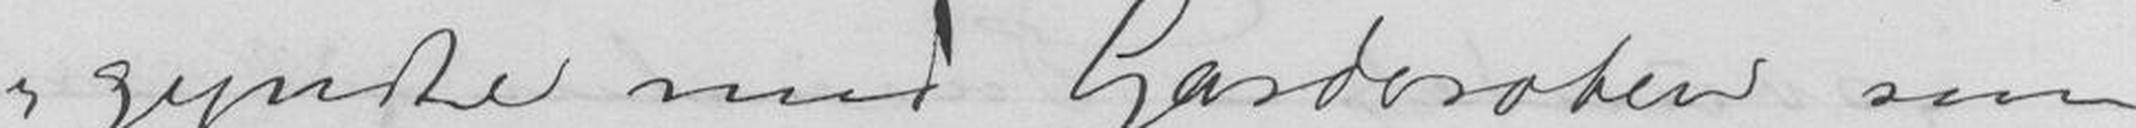gyndte med Garderoben som
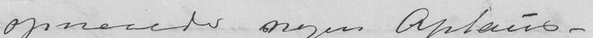opnaaede nogen Aplaus -
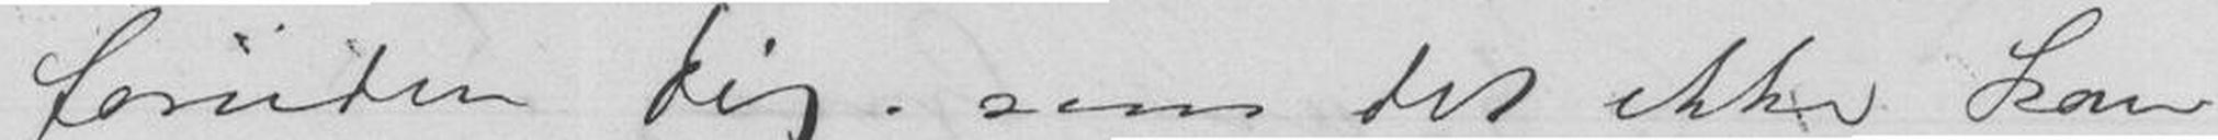foruden Dig, som det ikke kan
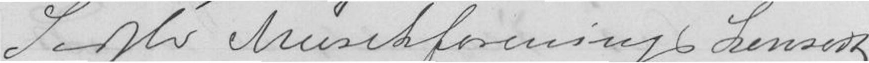Sidste Musikforenings konsert
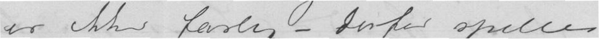er ikke farlig - Derfor spilles
